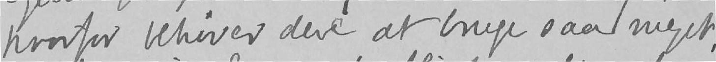hvorfor behøver dere at bruge saa meget,
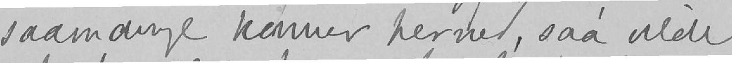saamange kommer herned, saa vilde
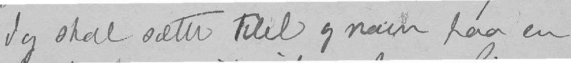Jeg skal sætte titel og navn paa en
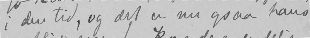i den tid, og det er nu ogsaa hans
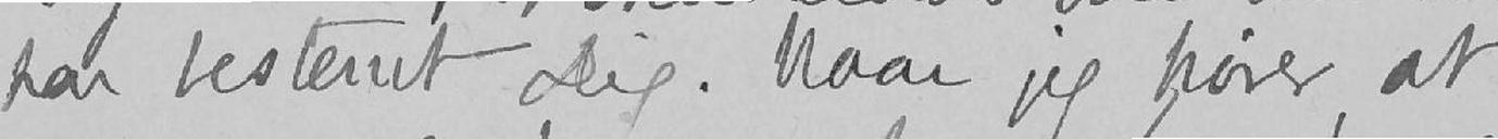har bestemt Dig. Naar jeg hører, at
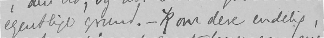egentlige grund. – Kom dere endelig,
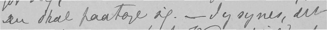Du skal paatage dig. – Jeg synes, det
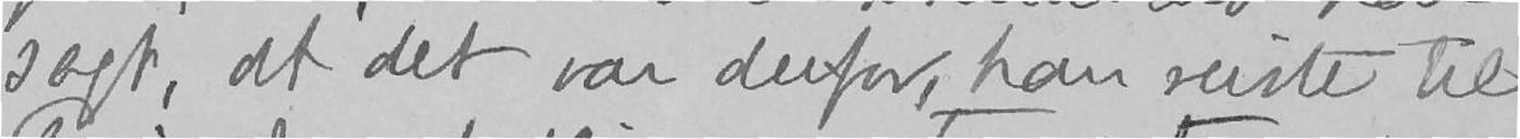sagt, at det var derfor, han reiste til
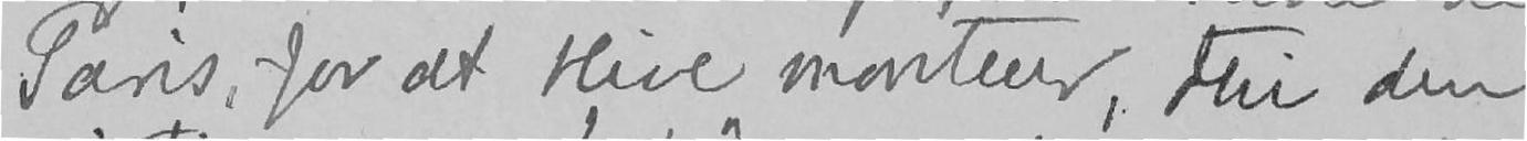Paris, for at blive monteur, thi den
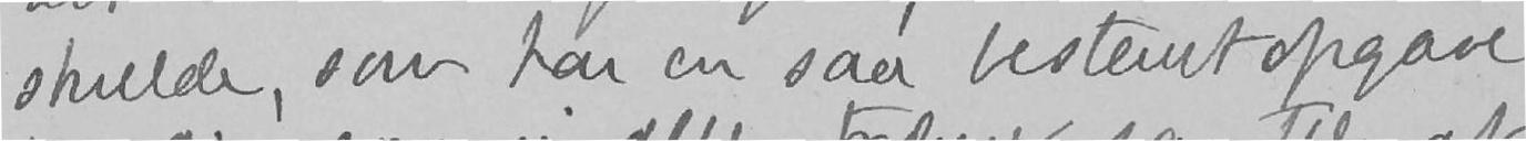skulde, som har en saa bestemt opgave
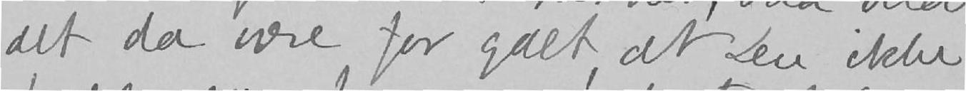det da være for galt, at Du ikke
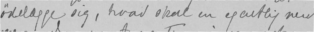ødelægge sig, hvad skal en egentlig med
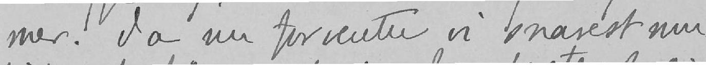mer. Ja nu forventer vi snarest mu-
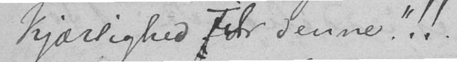Kjærlighed for denne"!!
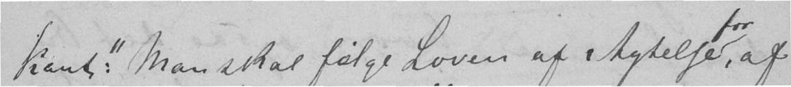Kant: "Man skal følge Loven af Agtelse, af
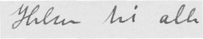Hilsen til alle
